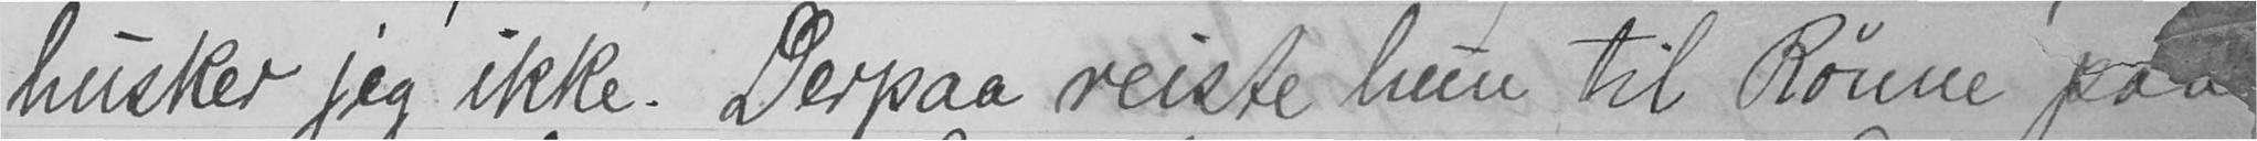husker jeg ikke. Derpaa reiste hun til Rønne paa
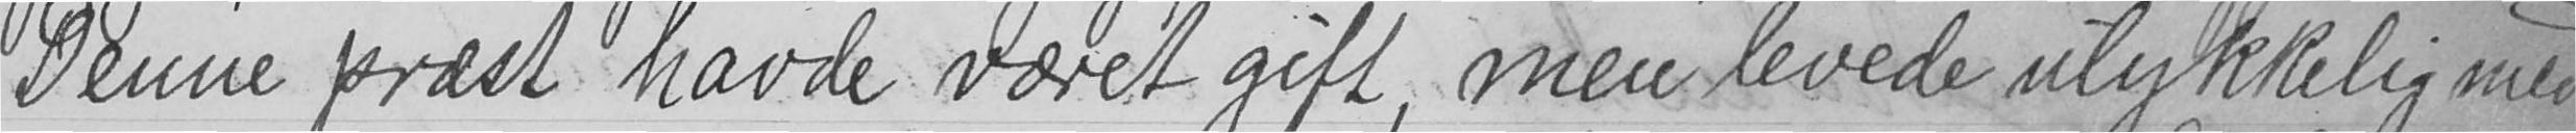Denne præst havde været gift, men levede ulykkelig med
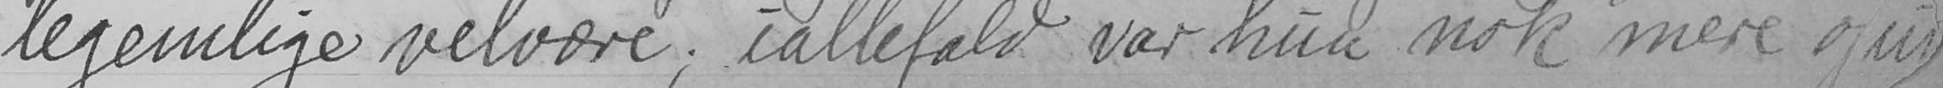legemlige velvære; iallefald. var hun nok mere op
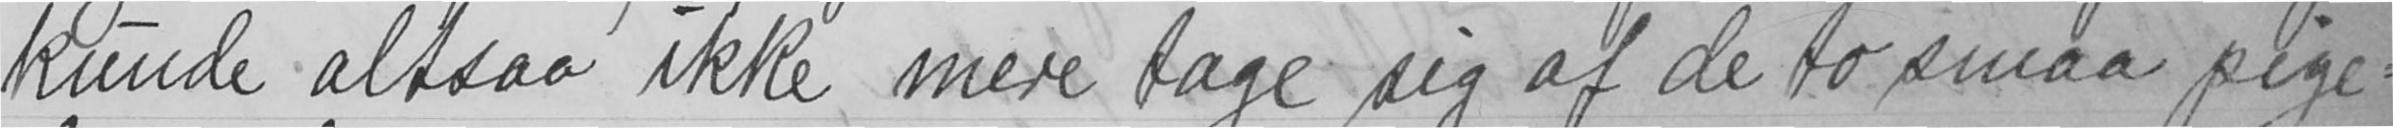kunde altsaa ikke mere tage sig af de to smaa pige-
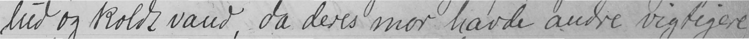lud og koldt vand, da deres mor havde andre vigtigere
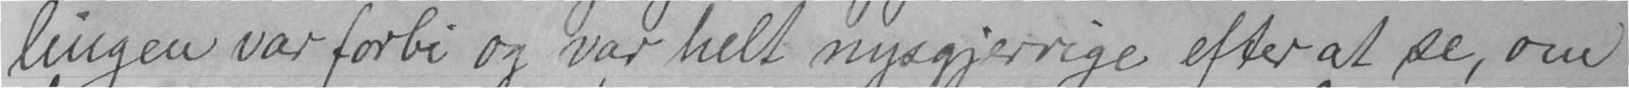lingen var forbi og var helt nysgjerrige efter at se, om
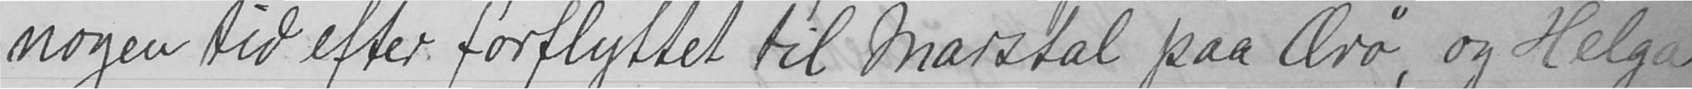nogen tid efter forflyttet til Marstal paa Ærø, og Helga
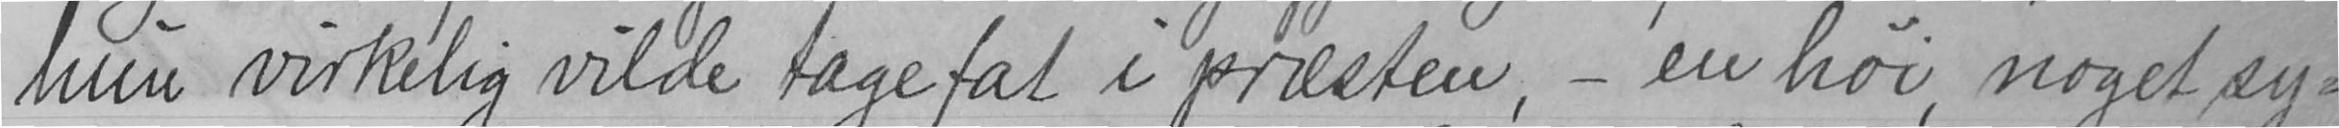hun virkelig vilde tage fat i præsten, - en høi, noget sy-
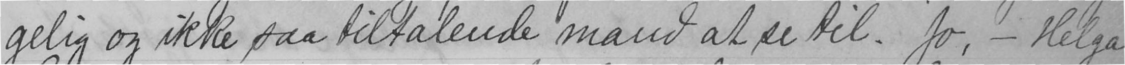gelig og ikke saa tiltalende mand at se til. Jo, - Helga
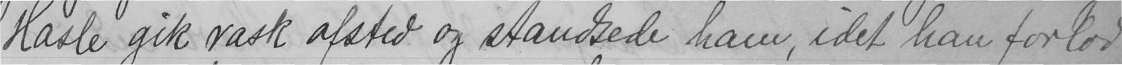Hasle gik rask afsted og standsede ham, idet han forlod
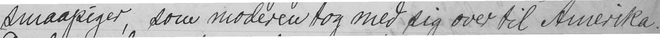smaapiger, som moderen tog med sig over til Amerika.
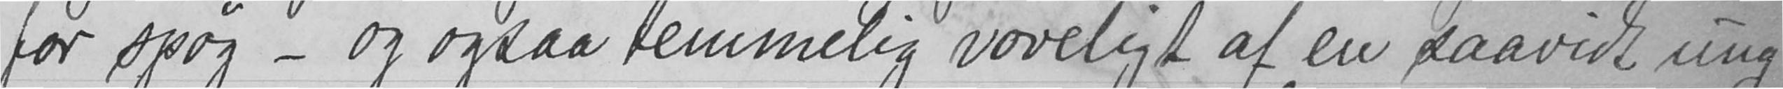for spøg - og ogsaa temmelig voveligt af en saavidt ung
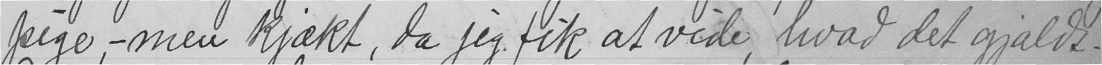pige, - men kjækt, da jeg fik at vide, hvad det gjaldt.
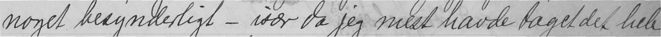noget besynderligt - især da jeg mest havde taget det hele
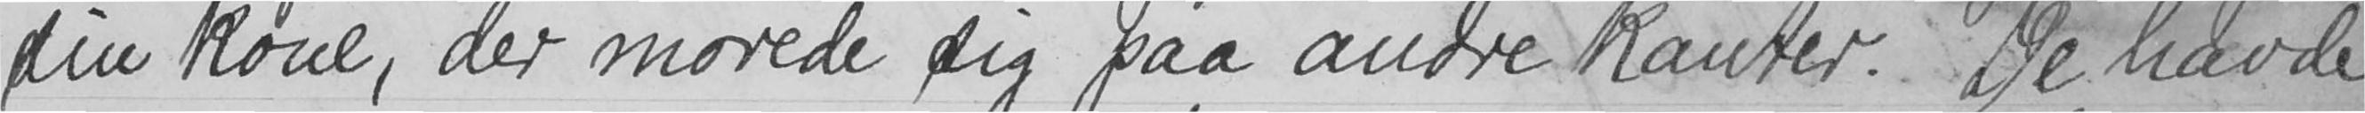sin kone, der morede sig paa andre kanter. De havde
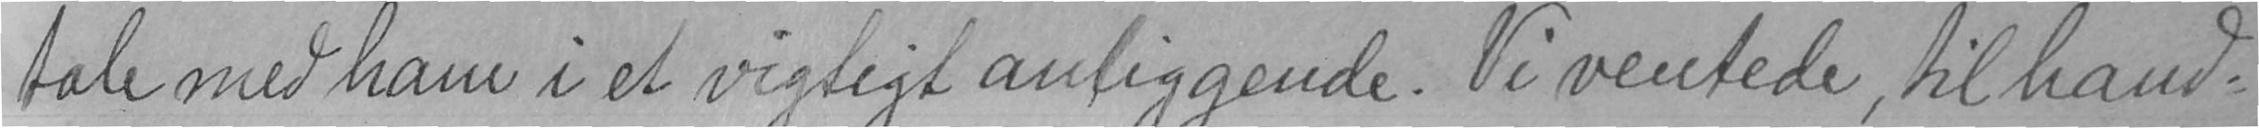tale med ham i et vigtigt anliggende. Vi ventede, til hand-
